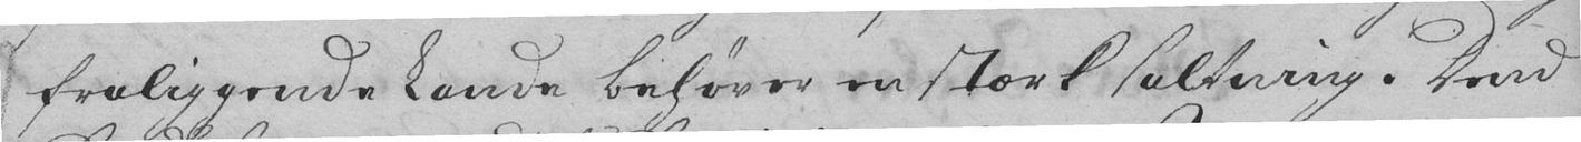fraliggende Lande behøver en stærk saltning. Dend
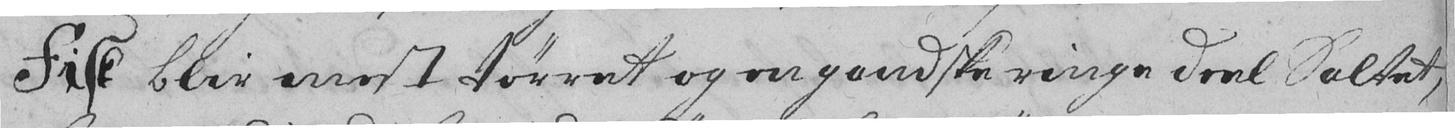Fisk blir mest tørret og en gandske ringe deel Saltet,
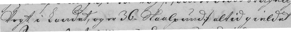Vegt i Landet, og er 36 Skaalpund :/ altid gieldet
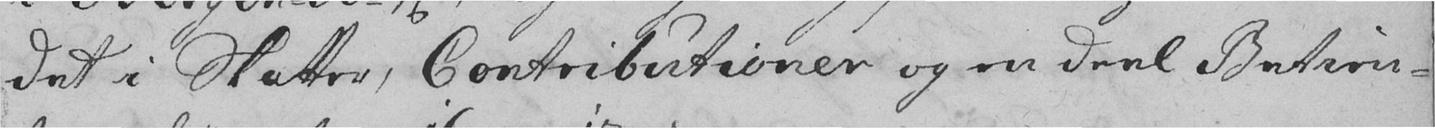det i Skatter, Contributioner og en deel Betien-
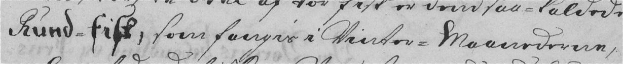Rund-fisk, som fangis i Vinter-Maanederne,
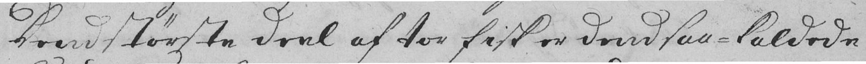Dend største deel af tor fisk er dend saa-kaldede
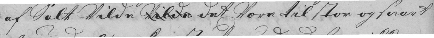af Salt Vilde  det være til stor og snart
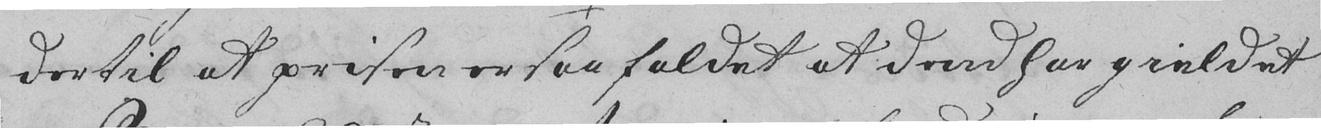dertil at prisen er saa faldet at dend har gieldet
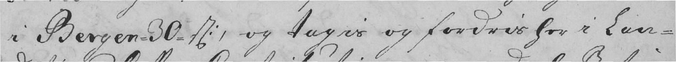i Bergen 30 sk., og tagis og fordris her i Lan-
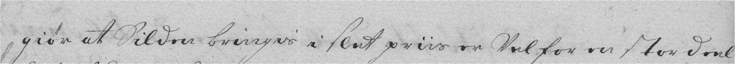giør at Silden bringis i slet priis er Vel for en stor deel
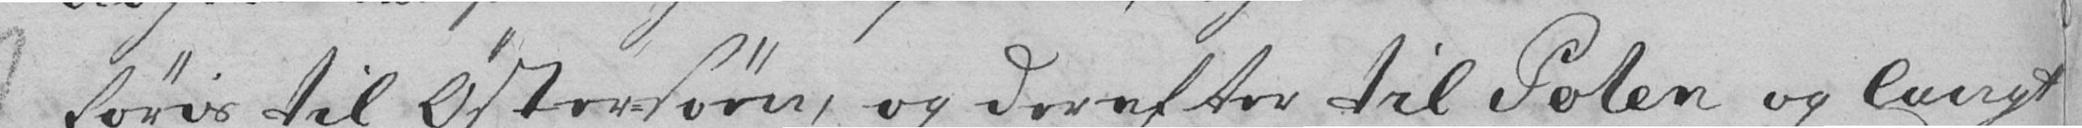føris til Øster-søen, og derefter til Polen og langt
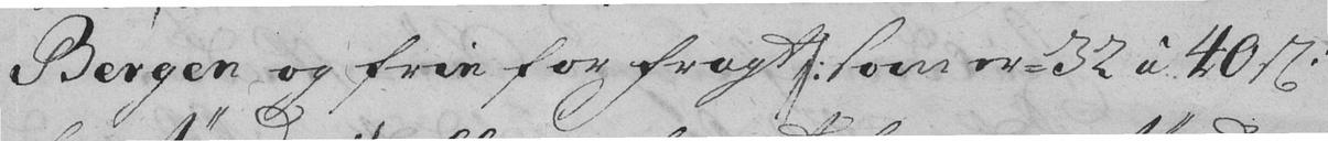Bergen og frie for Fragt /: som er 32 á 40 sk.
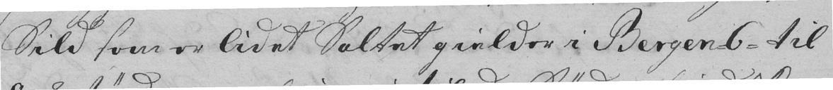Sild som er lidet Saltet gielder i Bergen 6 til
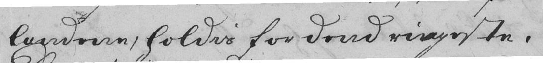landene, holdis for dend ringeste.
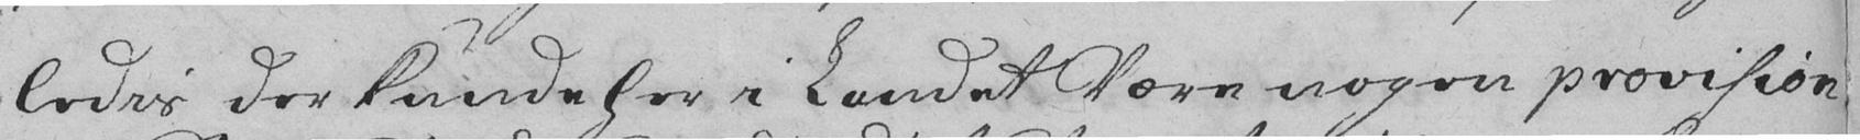ledis der kunde her i Landet Være nogen provision
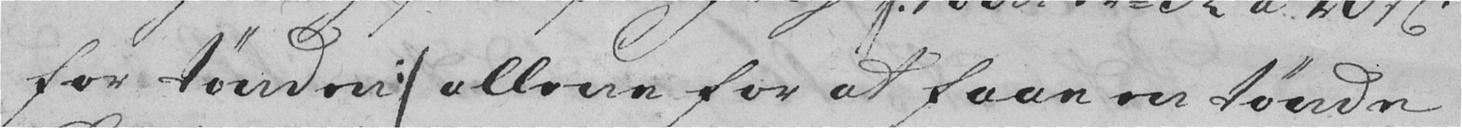for tønden :/ allene for at faae en tønde
